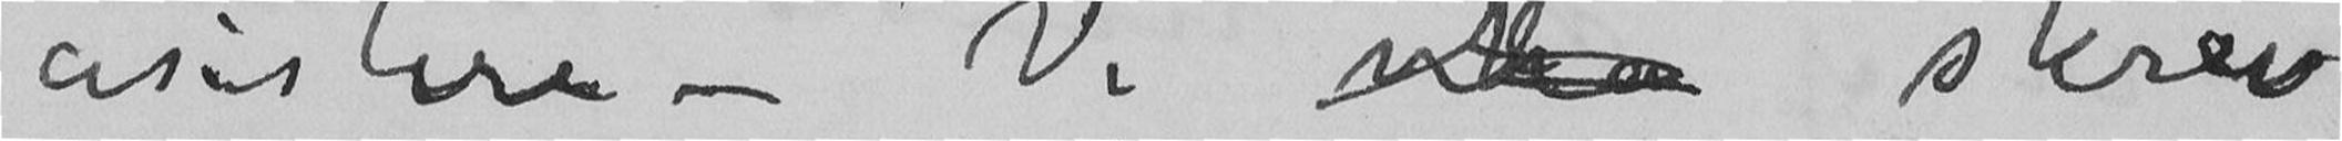asistere – Viskrev
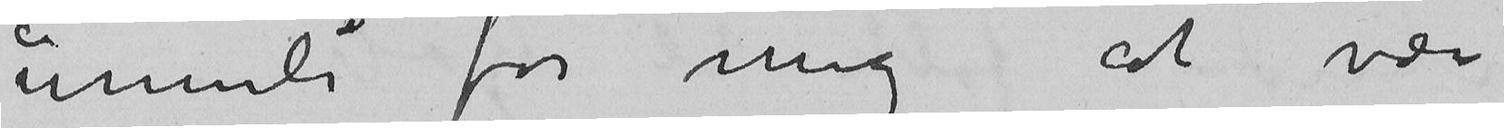umuli for mig at være
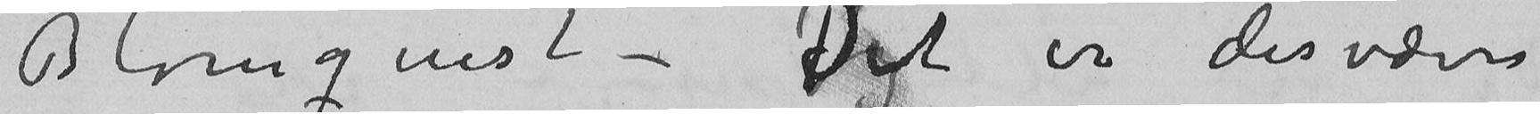Blomqvist – JegDet er desvære
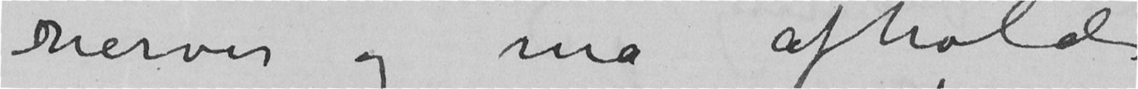nerver og må afholde
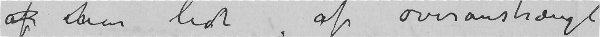af har lidt af overanstrængte
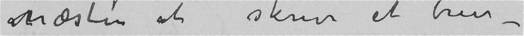næsten at skrive et brev –
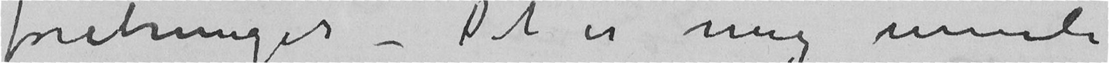foretninger – Det er mig umuli
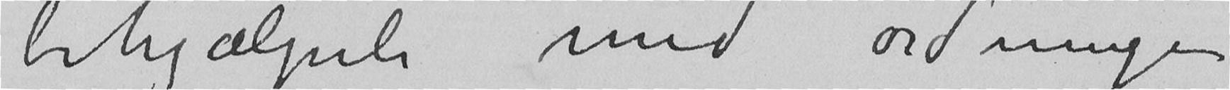behjælpeli med ordningen
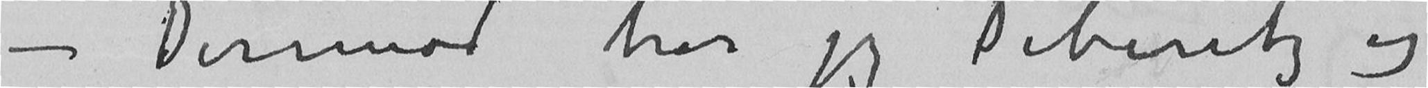– Derimod tror jeg Deberitz og
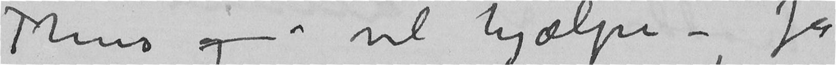Thiis og vil hjælpe – Ja
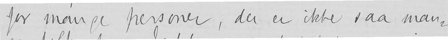for mange personer, der er ikke saa man-
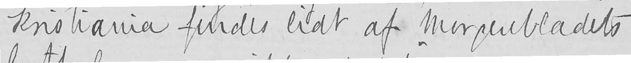Kristiania findes lidt af Morgenbladets
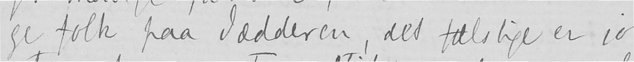ge folk paa Jædderen, det fælslige er jo
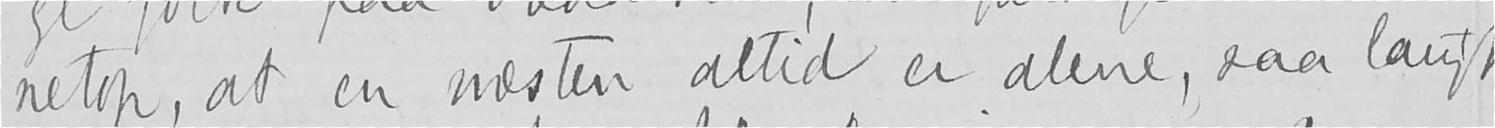netop, at en næsten altid er alene, saa langt
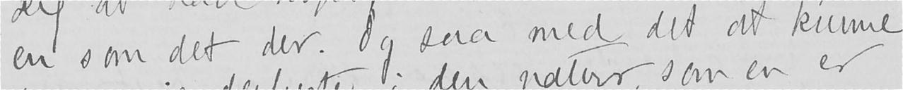en som det der. Og saa med det at kunne
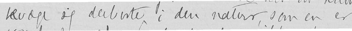bevæge sig derborte i den natur, som en er
